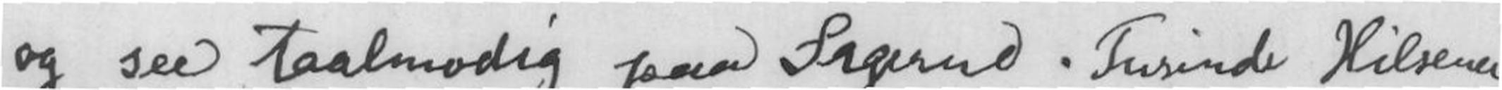og see taalmodig paa Sagerne. Tusinde Hilsener
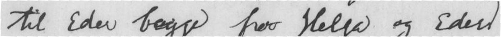til Eder begge fra Helga og Eders
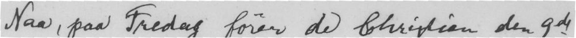Naa, paa Fredag fører de Christian den 9de
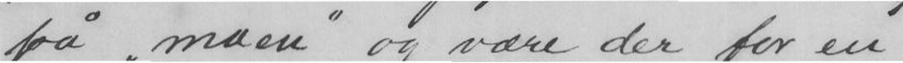på moen og være der for en
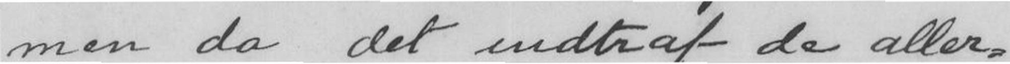men da det indtraf de aller-
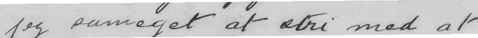jeg såmeget at stri med at
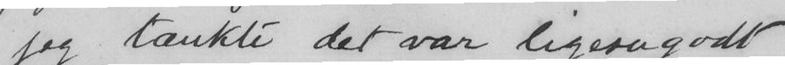jeg tænkte det var ligesågodt
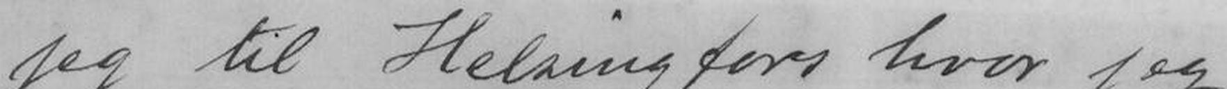jeg til Helsingfors hvor jeg
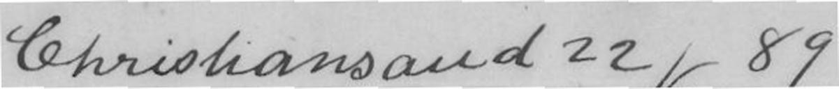Christiansand 22/6 89
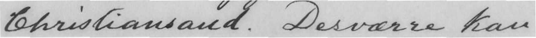Christiansand. Desværre kan
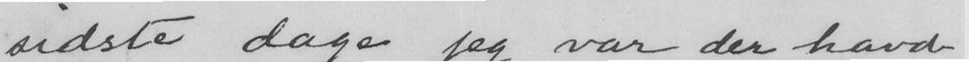sidste dage jeg var der havde
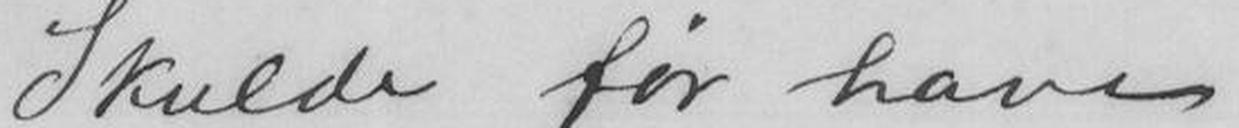Skulde før have
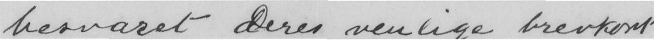besvaret Deres venlige brevkort
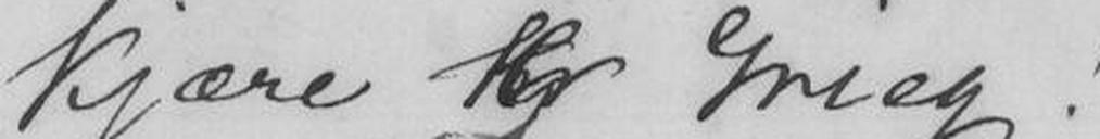Kjære overskrevet: G Hr Grieg!
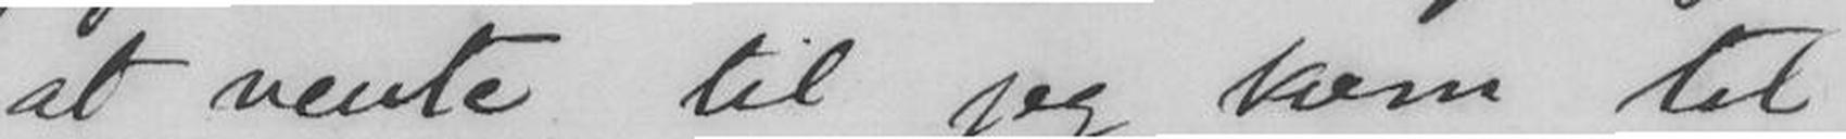at vente til jeg kom til
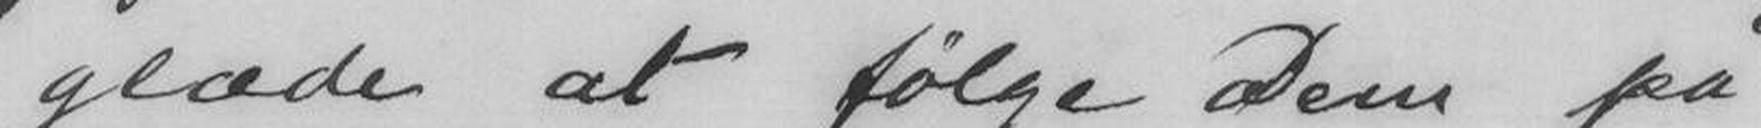glæde at følge Dem på
